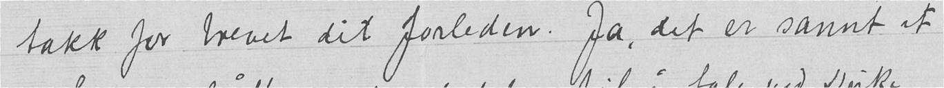takk for brevet dit forleden. Ja, det er sannt at
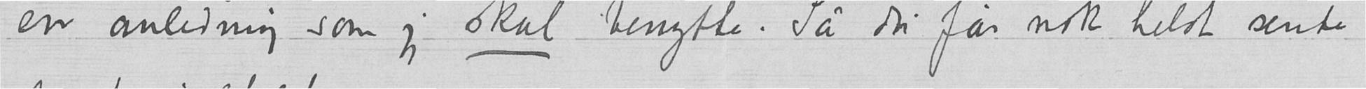en anledning som jeg skal benytte. Så du får nok helst sende
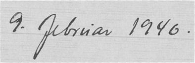9. februar 1940.
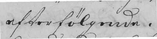efterfølgende.
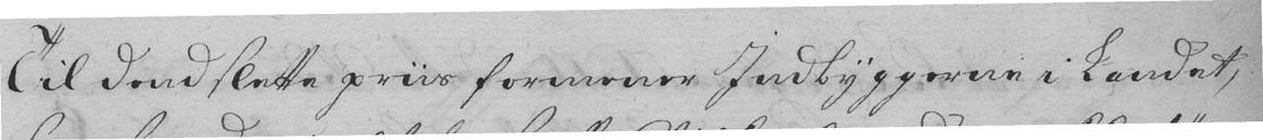Til dend slette priis formener Indbyggerne i Landet,
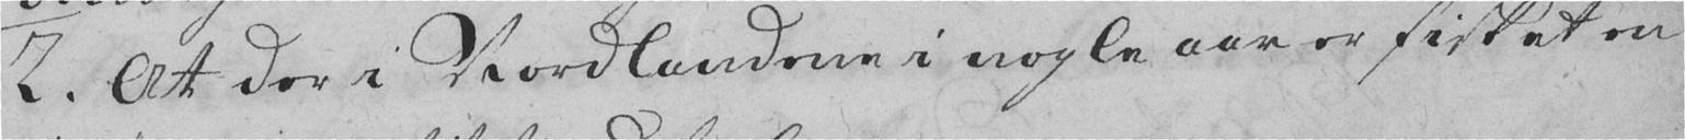2. At der i Nordlandene i nogle aar er fisket en
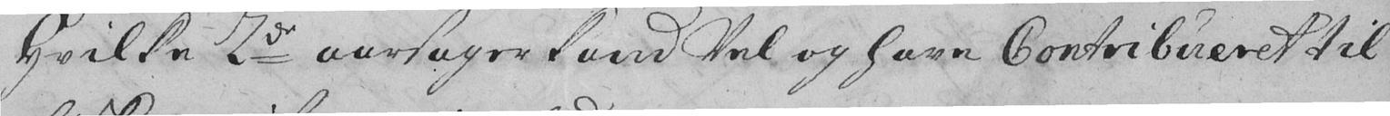Hvilke 2de aarsager kand Vel og have Contribueret til
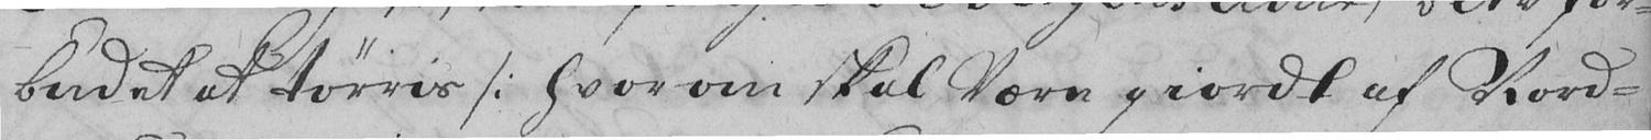budet at tørris /: hvorom skal være giordt af Nord-
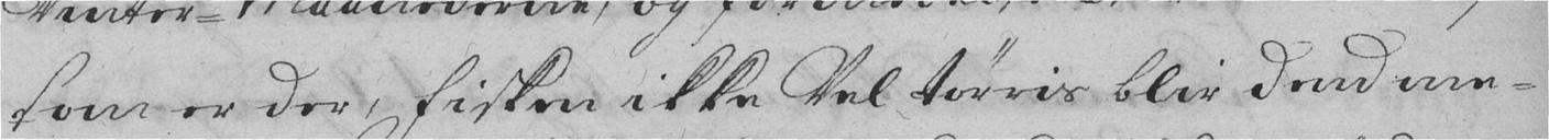som er der, Fisken ikke Vel tørris blir dend me-
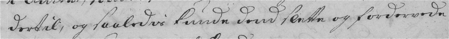dertil, og saaledis kunde dend slette og fordervede
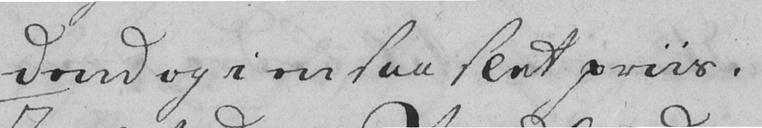dend og i en saa slet priis.
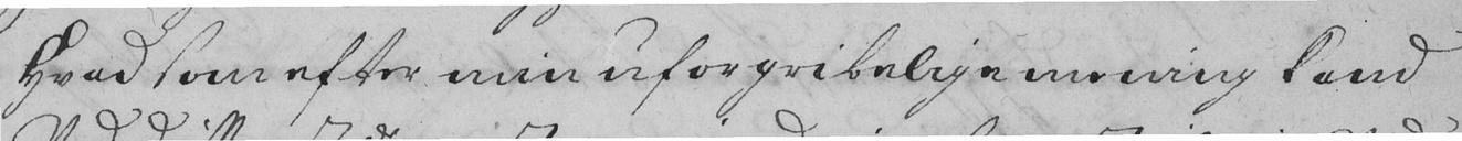Hvad som efter min uforgribelige mening kand
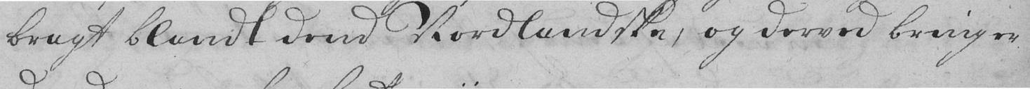bragt blandt dend Nordlandske, og derved bringer
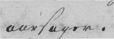aarsager.
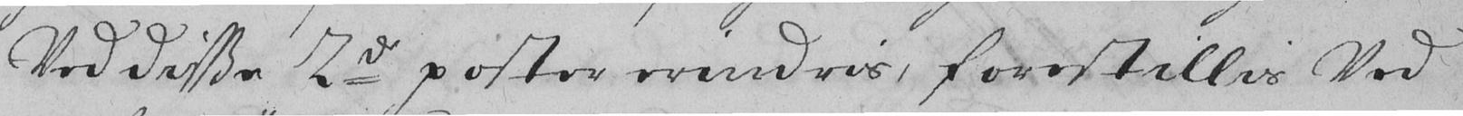Ved disse 2de poster erindris, forestillis Ved
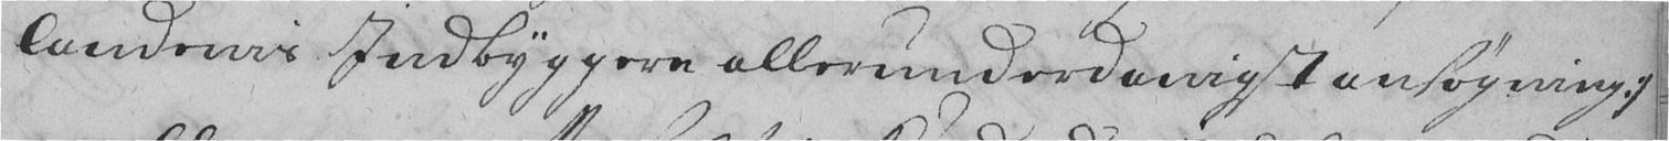landenis Indbyggere allerunderdanigst ansøgning :/
